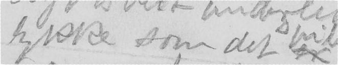lykke som det er
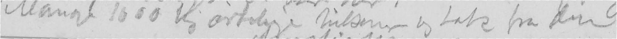Mange 1000 hjærtelige hilsener og tak fra din
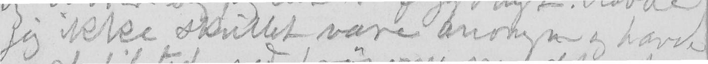jeg ikke skullet være anonym og havde
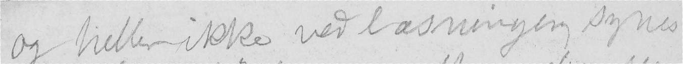og heller ikke ved læsningen synes
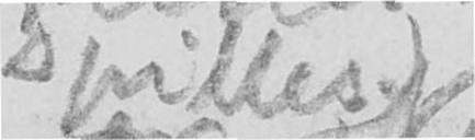spilles.
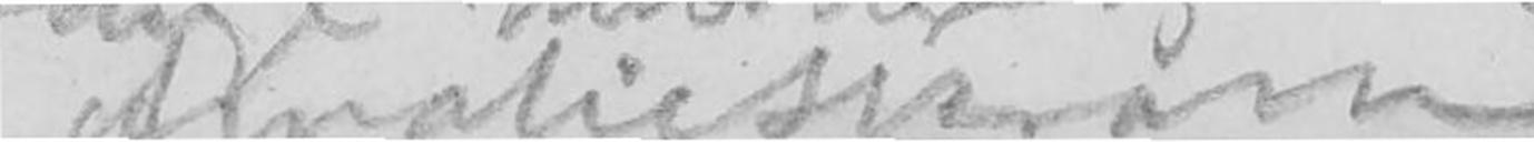Amalie Skram
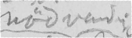nødvendig
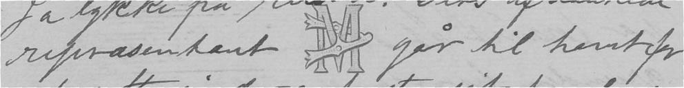repræsentant går til havet for
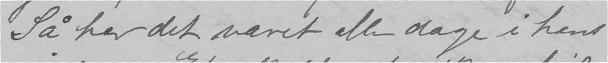Så har det været alle dage i hans
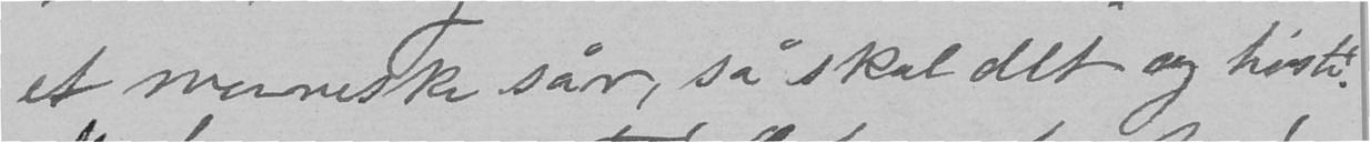et menneske sår, så skal det og høste".
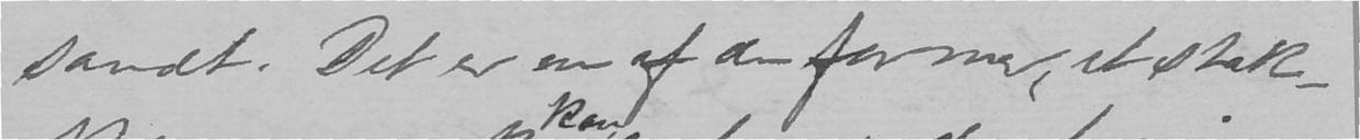sandt. Det er en af de former, et stak-
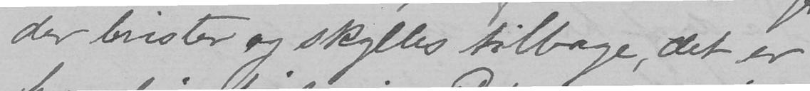der brister og skylles tilbage, det er
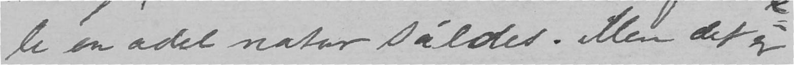le en ædel natur således. Men det er
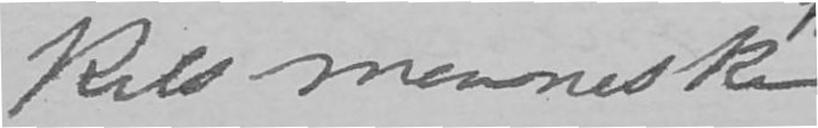kels menneske
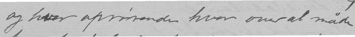og hvor oprørende hvor over al måde
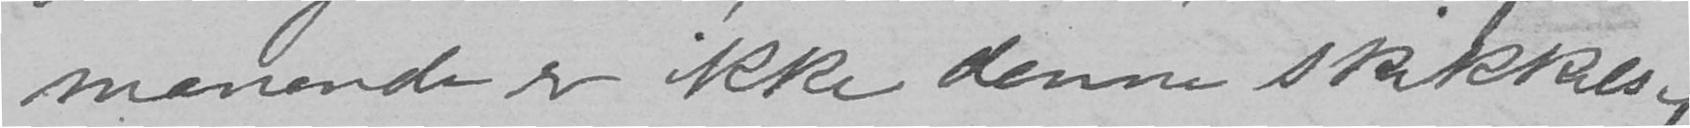manende er ikke denne skikkelse,
